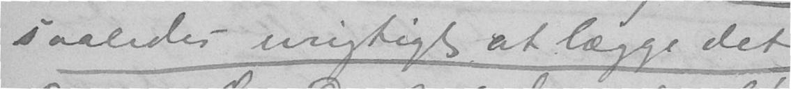saaledes urigtigt at lægge det
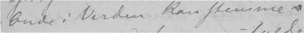Onde i Verden kan stemme
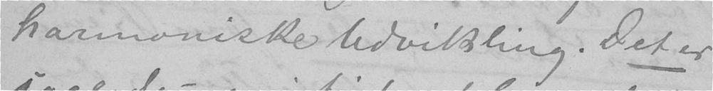harmoniske Udvikling. Det er
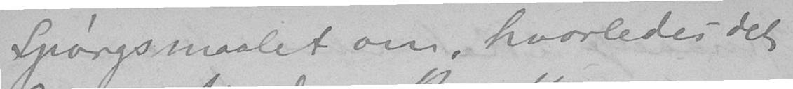Spørgsmaalet om, hvorledes det
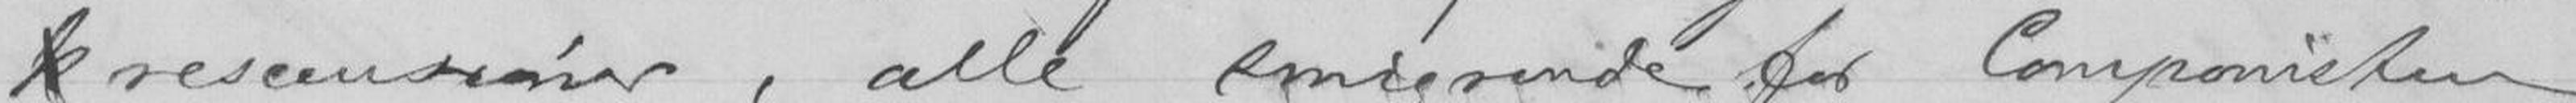krescensioner, alle smigrende for Componisten
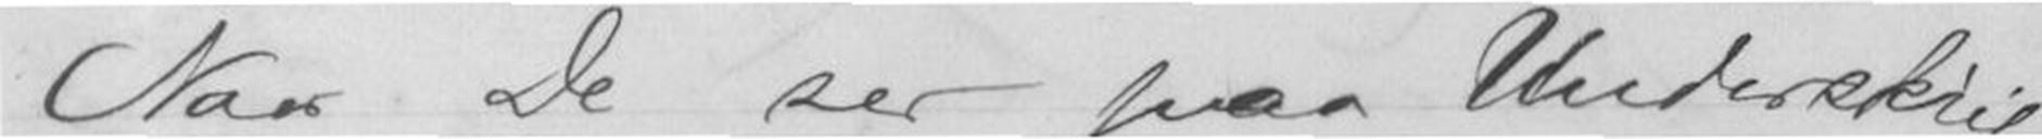Naar De ser paa Underskrif
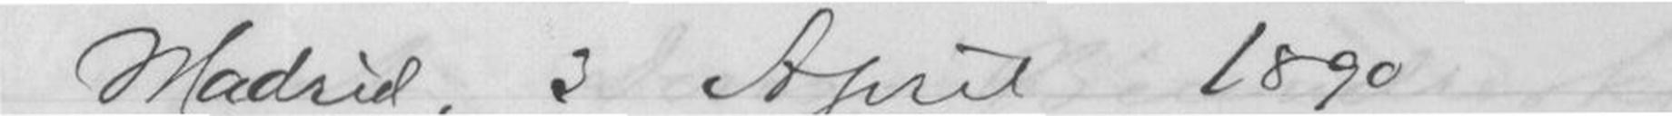Madrid, 3 April 1890
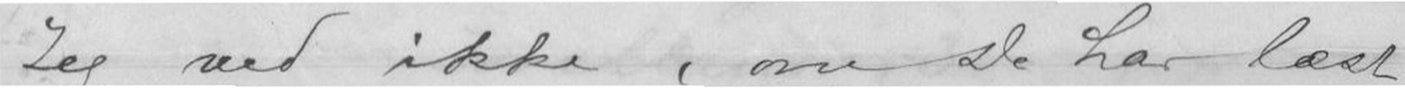Jeg ved ikke, om De har læst
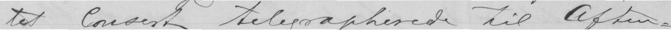tet Consert telegrapherede til Aften-
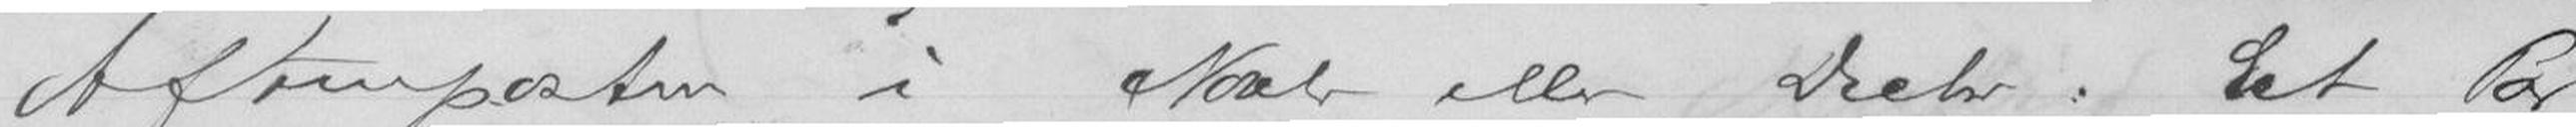Aftenposten i Novbr eller Decbr. Et Par
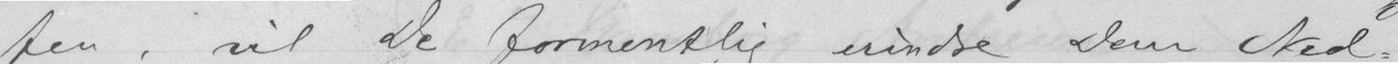ten, vil De formentlig erindre Dem Ned-
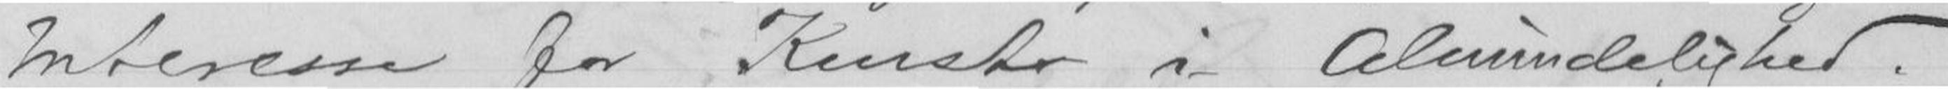Interesse for Kunst i Almindelighed.
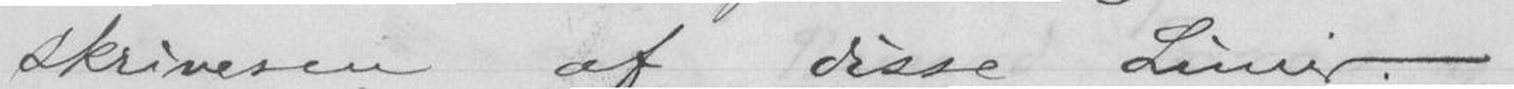skriveren af disse Linier.
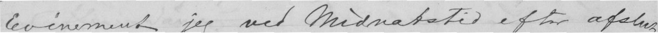Evinement jeg ved Midnatstid efter afslut-
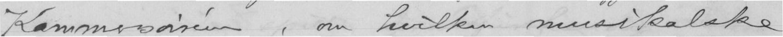Kammersoiréen, om hvilken musikalske
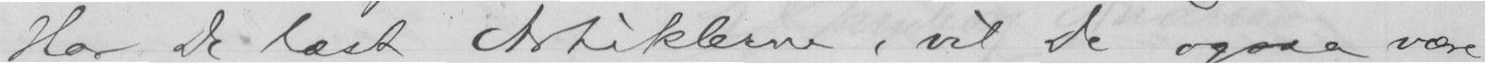Har De læst Artiklerne, vil De ogsaa være
